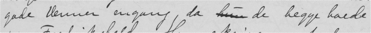gode Venner engang, da  de begge boede
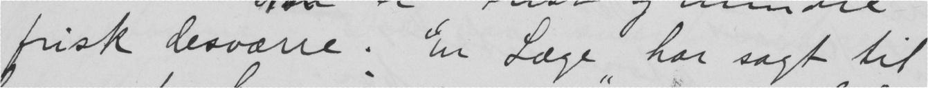frisk desværre. En Læge har sagt til
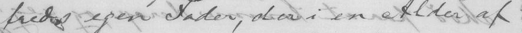freds egen Fader, der i en Alder af
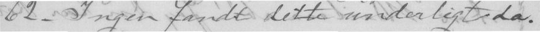62. Ingen fandt dette underligt da.
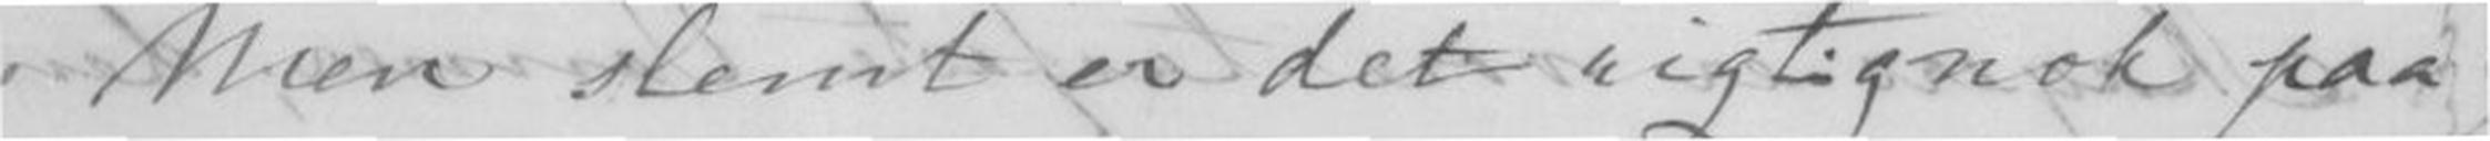Men slemt er det rigtignok paa
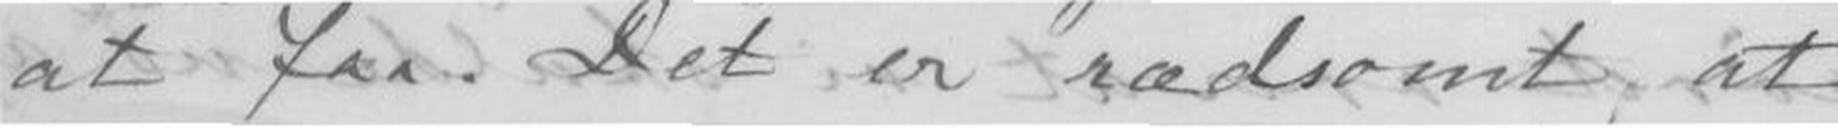at faa. Det er rædsomt at
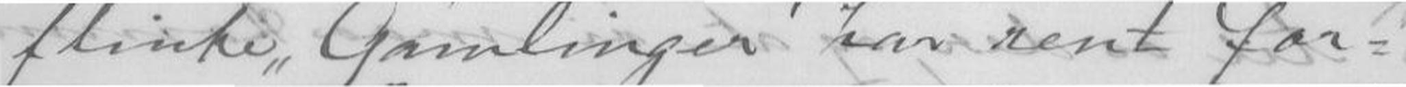flinke Gamlinger har rent for-
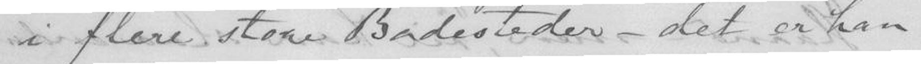i flere store Badesteder - det er han
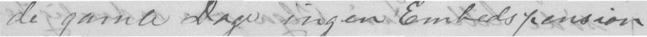de gamle Dage ingen Embedspension
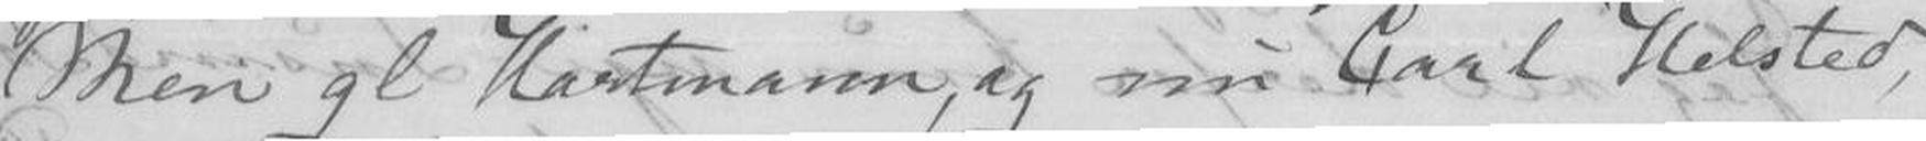Men gl Hartmann, og nu Carl Helsted,
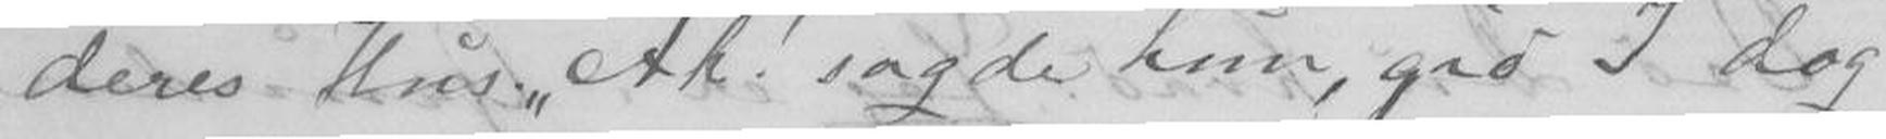deres Hus. Ak! sagde hun, gid I dog
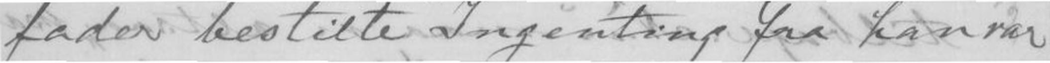fader bestilte Ingenting saa han var
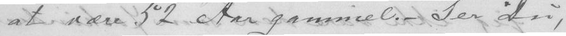at være 52 Aar gammel. - Ser Du,
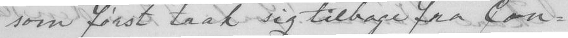som først trak sig tilbage fra Con-
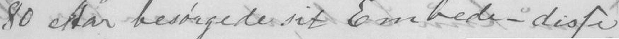80 Aar besørgede sit Embede - disse
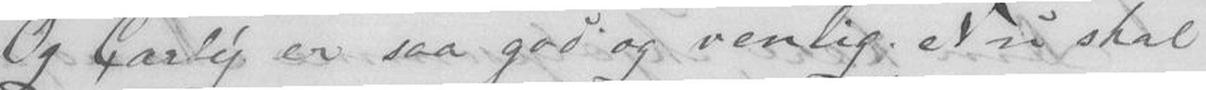Og Carly er saa god og venlig; e. nu skal
